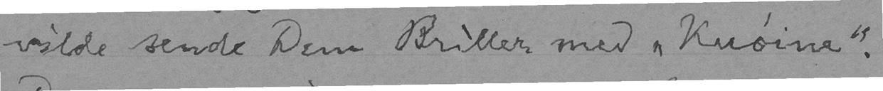vilde sende Dem Briller med "Kuøine".
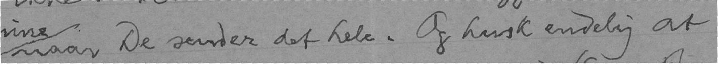naar De sender det hele. Og husk endelig at
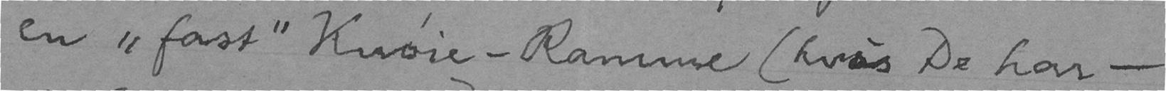en "fast" Kuøie-Ramme (hvis De har -
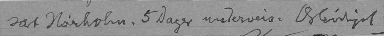sat Nørholm. 5 Dager underveis. Ærbødigst
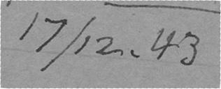17/12. 43.
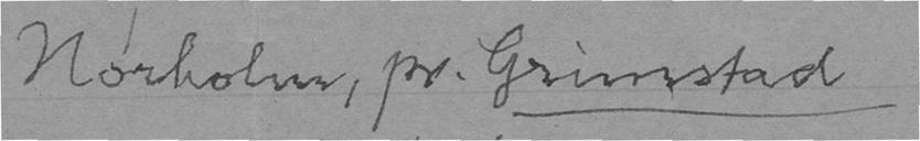Nørholm, pr. Grimstad
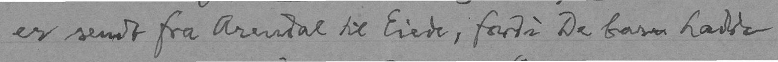er sendt fra Arendal til Eiede, fordi De bare hadde
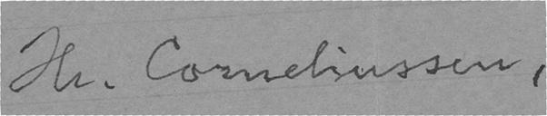Hr. Corneliussen,
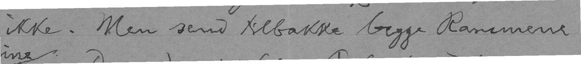ikke. Men send tilbakke begge Rammene
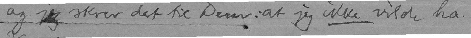og jeg skrev det til Dem: at jeg ikke vilde ha
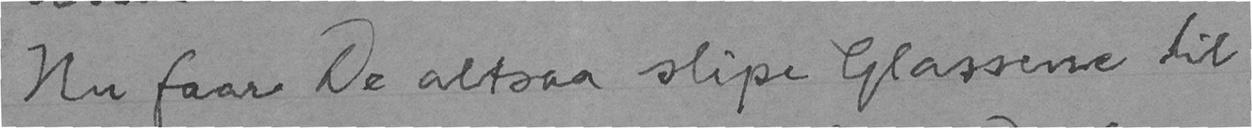Nu faar De altsaa slipe Glassene til
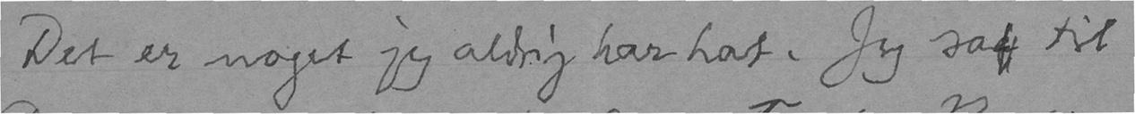Det er noget jeg aldrig har hat. Jeg saa til
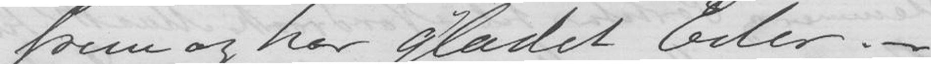frem og har glædet Eder. -
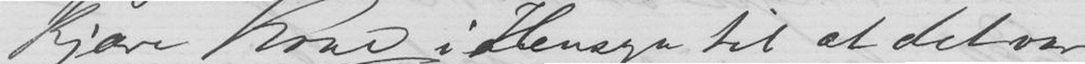Kjære Kone i Hensyn til at det var
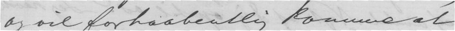og vil forhaabentlig komme at
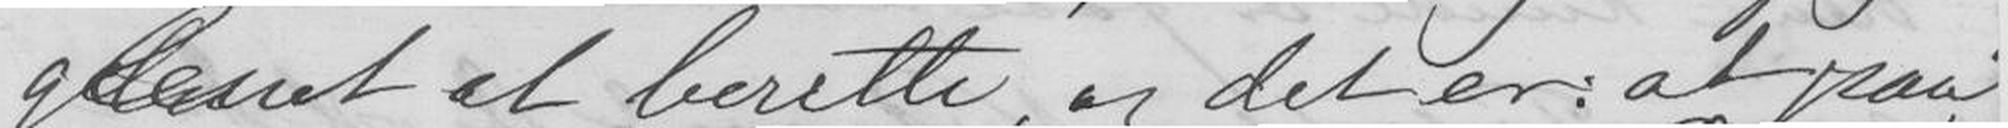glemt at berette, og det er: at paa
